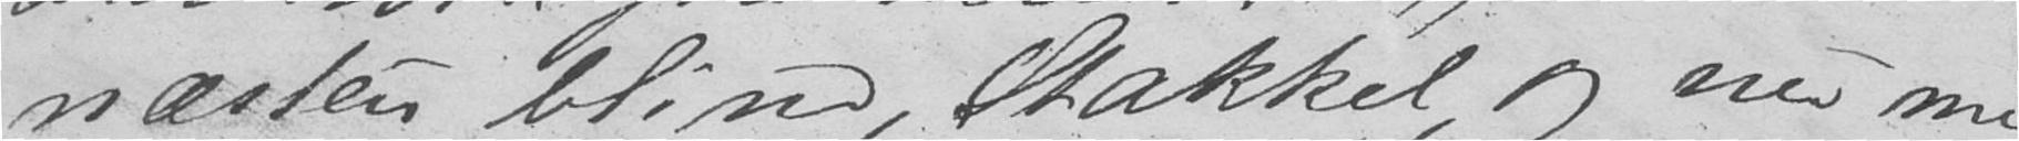næsten blind, Stakkel, og nu me-
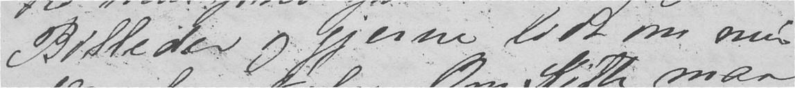Billeder og gjerne lidt om min
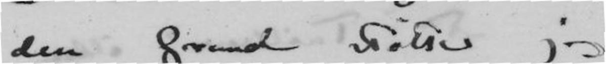den grund støtter vi
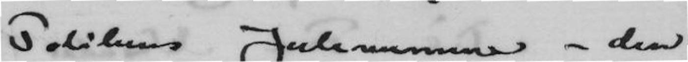Polikens Julenummer - den
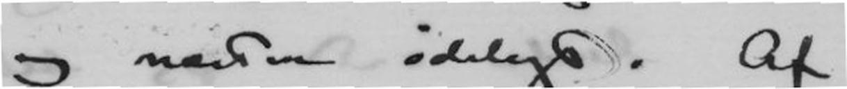og næsten ødelagt. Af
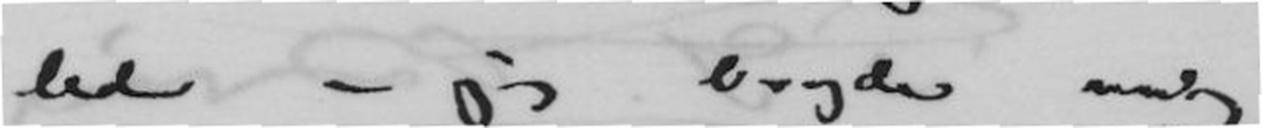led - jeg b.gde mig
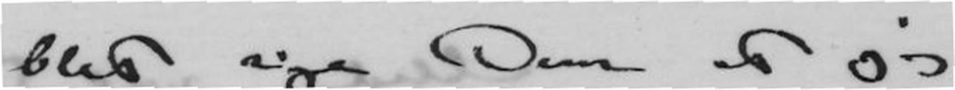blot sige Dem at vi
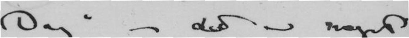Dag" - det - er noget
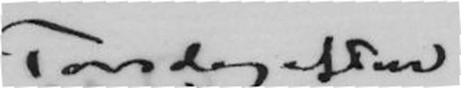Torsdag aften
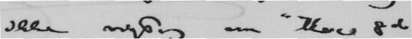ikke nytig om "Men god
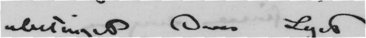ubetinget Dem Lyst
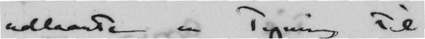udlaandte en Tigning til
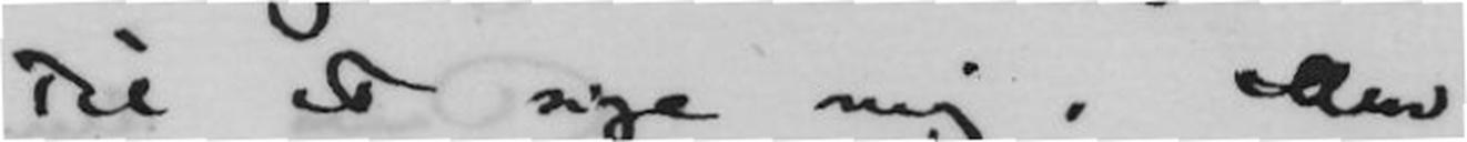til at sige mig. Men
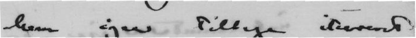kom igaar tilbage iturevet
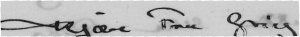Kjære Fru Grieg
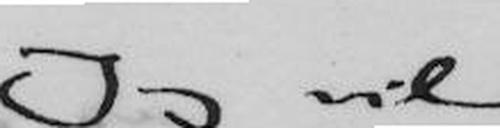Jeg vil
